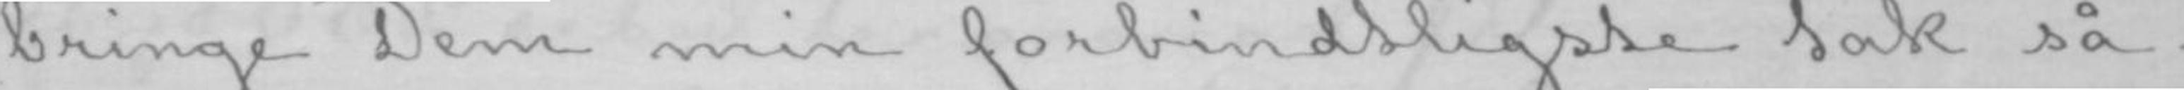bringe Dem min forbindtligste tak så-
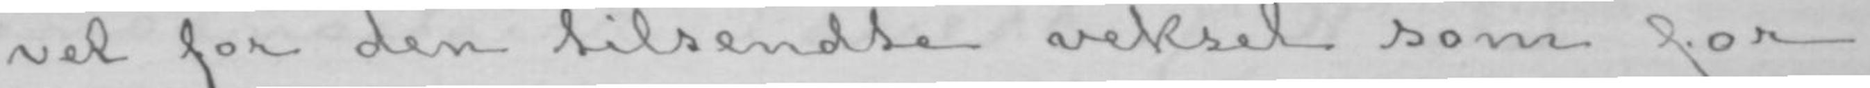vel for den tilsendte veksel som for
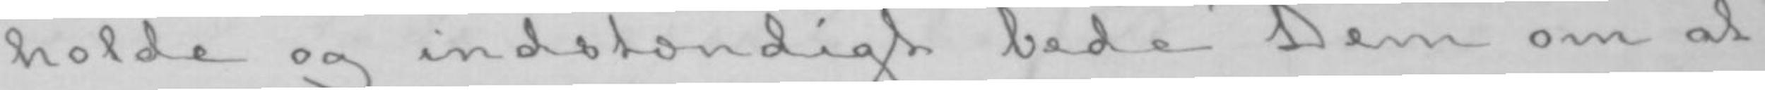holde og indstændigt bede Dem om at
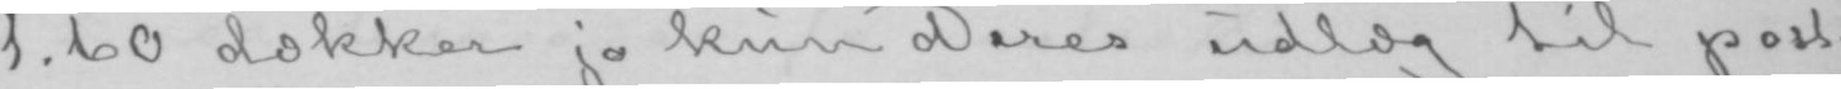1.60 dækker jo kun Deres udlæg til post-
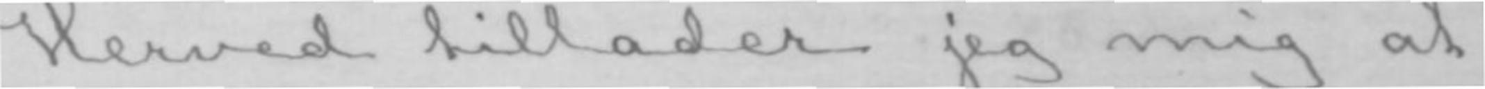Herved tillader jeg mig at
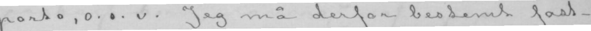porto, o. s. v. Jeg må derfor bestemt fast-
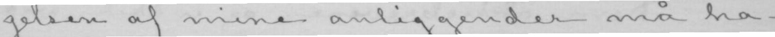gelsen af mine anliggender må ha-
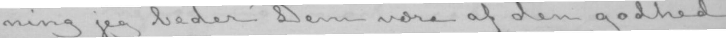ning jeg beder Dem være af den godhed
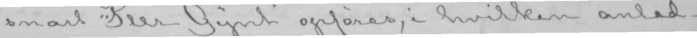snart «Peer Gynt» opføres, i hvilken anled-
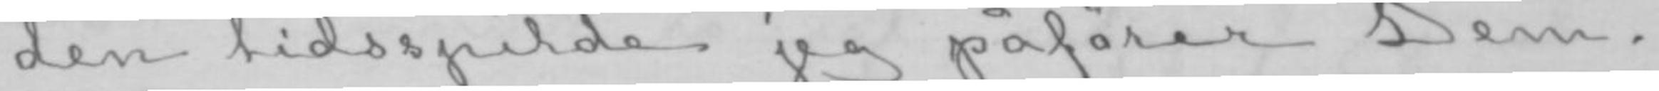den tidsspilde jeg påfører Dem.
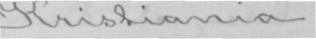Kristiania.
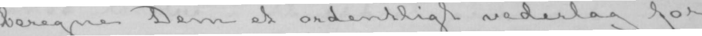beregne Dem et ordentligt vederlag for
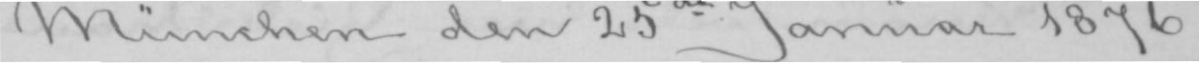München den 25de Januar 1876.
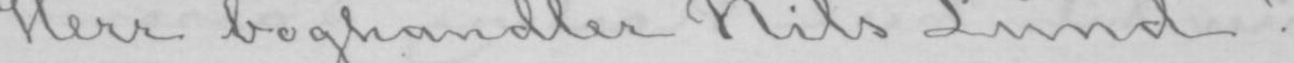Herr boghandler Nils Lund!
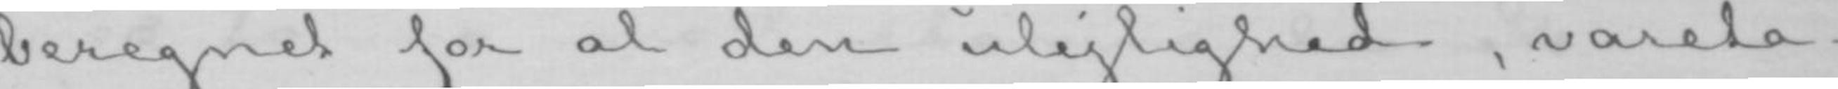beregnet for al den ulejlighed, vareta-
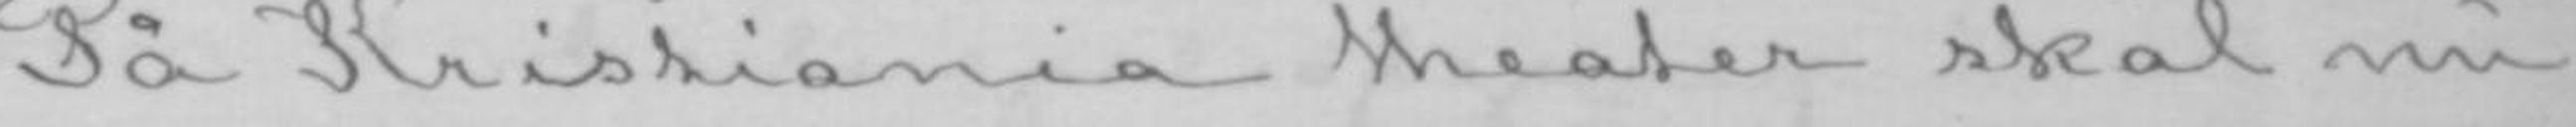På Kristiania theater skal nu
